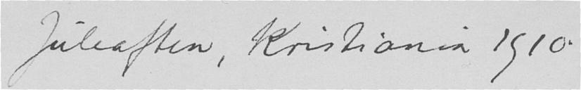Juleaften, Kristiania 1910.
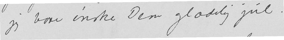jeg bare ønske Dem glædelig jul.
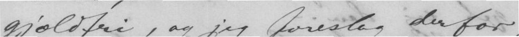gjældfri, og jeg foreslog derfor,
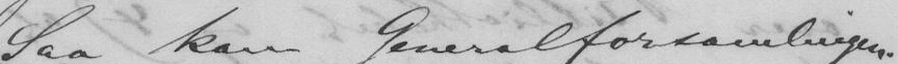Saa kom Generalforsamlingen.
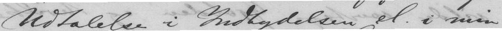Udtalelse i Indbydelsen el. i min
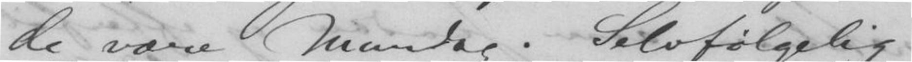de være Mandag. Selvfølgelig
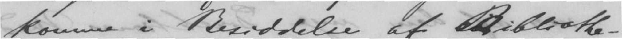komme i Besiddelse af Bibliothe-
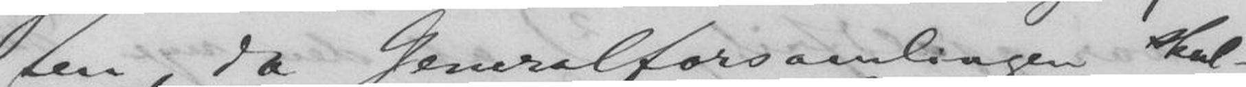ten, da Generalforsamlingen skul-
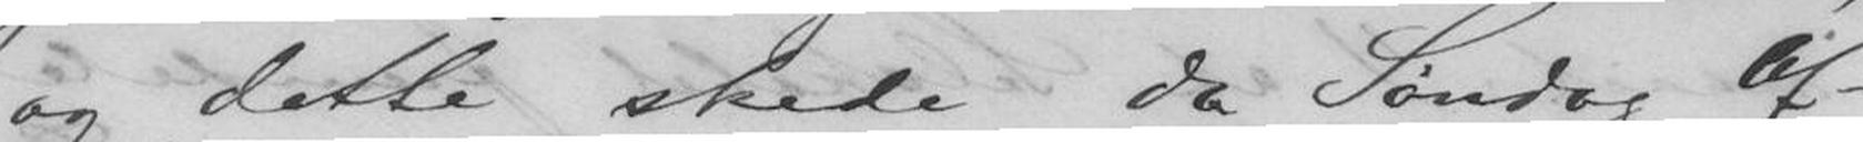og dette skedede da Søndag Af-
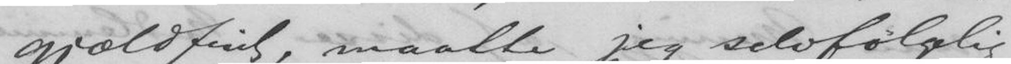gjældfrit, maatte jeg selvfølgelig
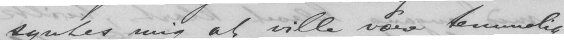syntes mig at ville være temmelig
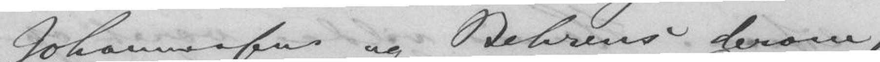Johannessen og Behrens derom,
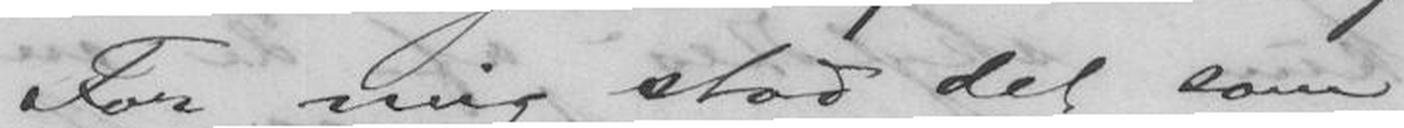For mig stod det som
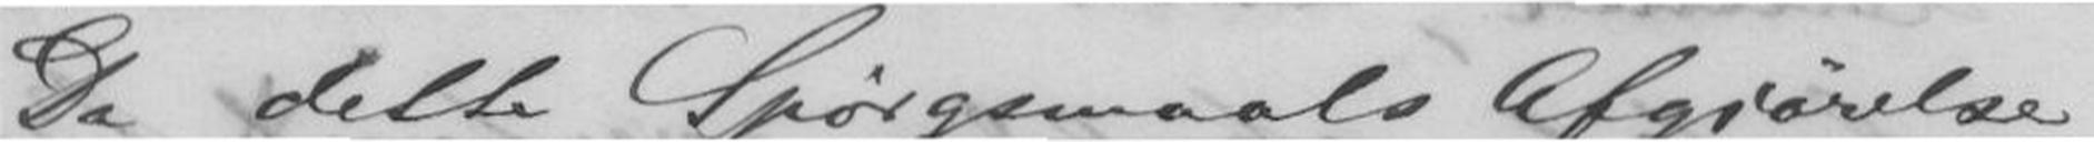Da dette Spørgsmaals Afgjørelse
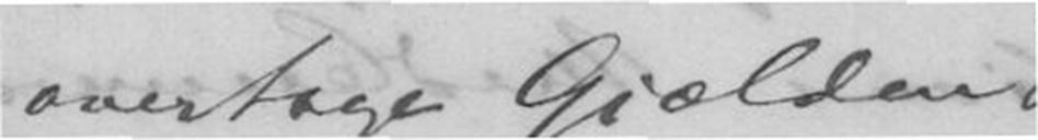overtage Gjælden
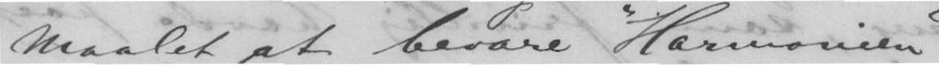Maalet at bevare "Harmonien"
